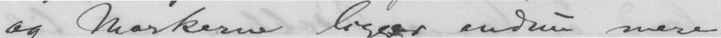og Markerne ligger endnu mere
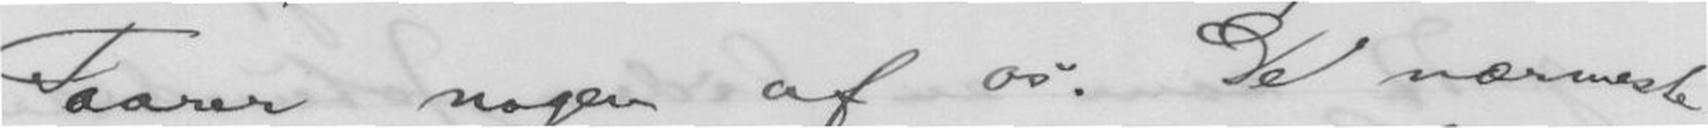Taarer nogen af os. De nærmeste
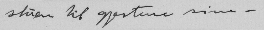stuen til gjestene sine -
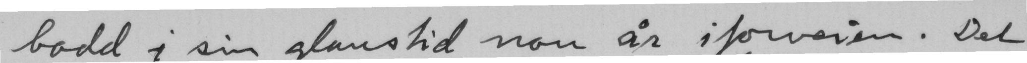bodd i sin glanstid noen år iforveien. Det
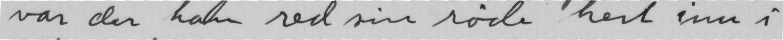var der han red sin røde hest inn i
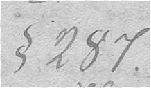§ 287.
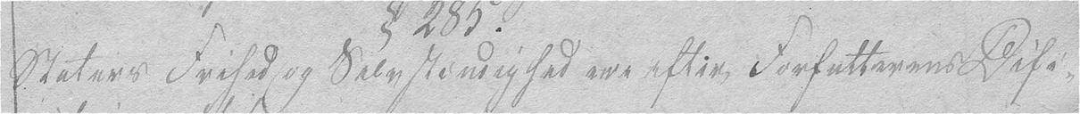Staters Frihed og Selvstændighed ere efter Forfatterens Defi-
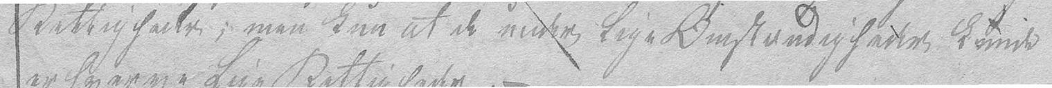Rettigheder, men kun at de under lige Omstændigheder kunde
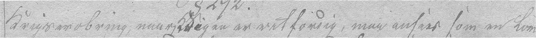Krigserobring naar Krigen er retfærdig, maa ansees som en lov-
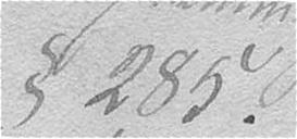§ 285.
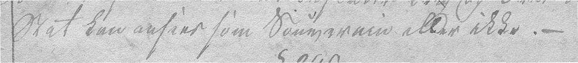Stat kan ansees som Souverain eller ikke. –
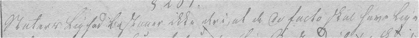Staters Lighed bestaaer ikke deri, at de de facto skal have lige
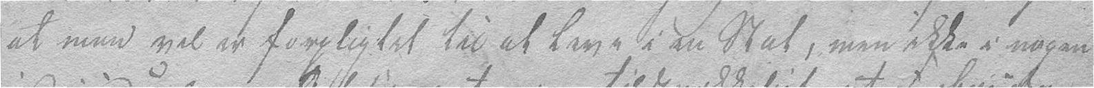at man vel er forpligtet til at leve i en Stat, men ikke i nogen
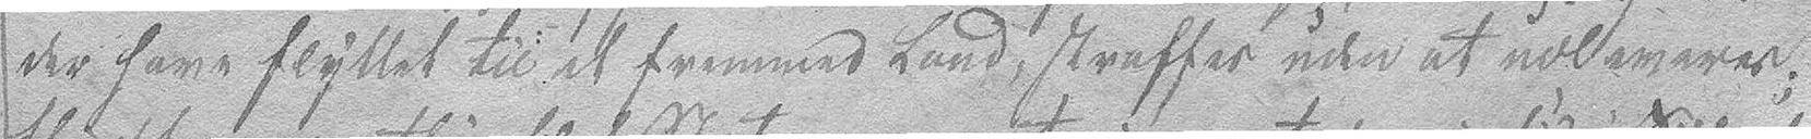der have flyttet til et fremmed Land straffes uden at udleveres;
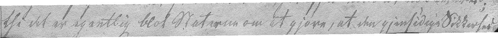thi det er egentlig blot Staterne om at gjøre, at den gjensidige Sikkerhed
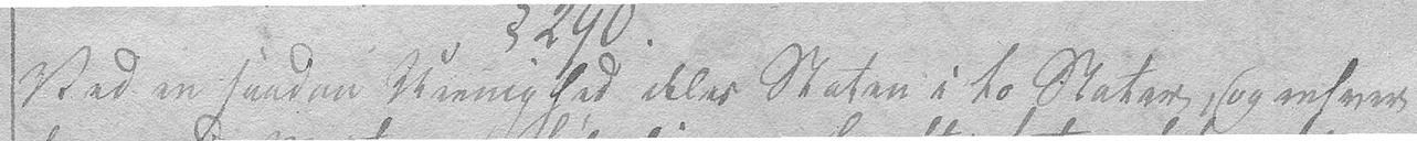Ved en saadan Uenighed deles Staten i to Stater, og enhver
